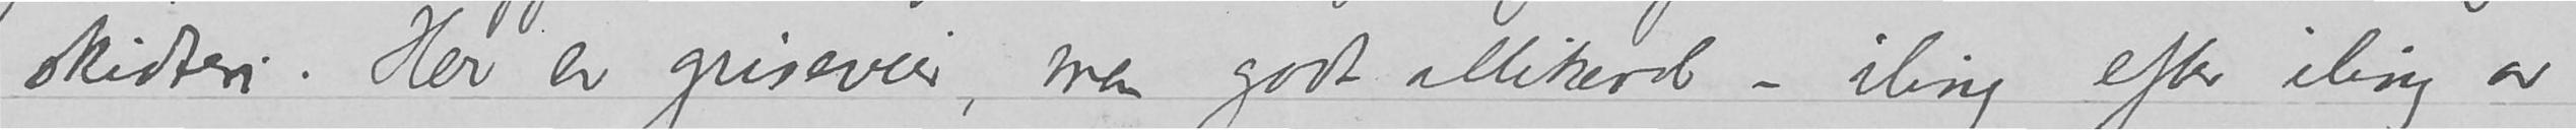skidteri. Her er griseveir, men godt allikevel – iling efter iling av
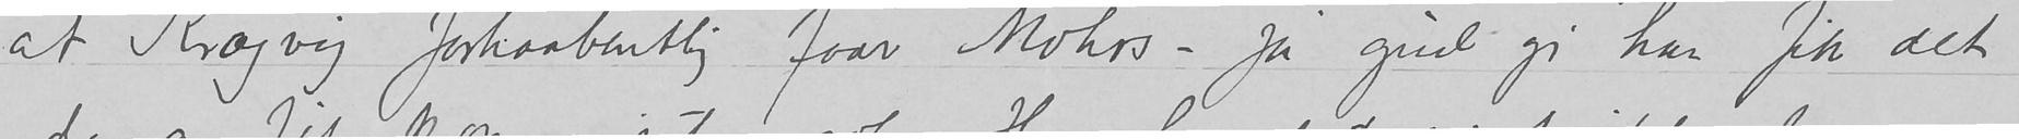at Krogvig forhaabentlig faar Mohrs – ta gud gi han fik det
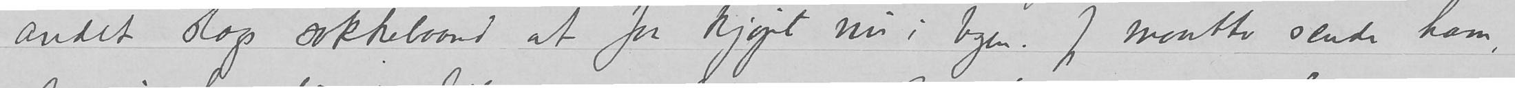andet slags sokkelvand at faa kjøpt nu i byen. Jeg maatte sende ham,
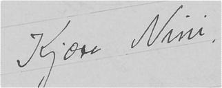Kjære Nini,
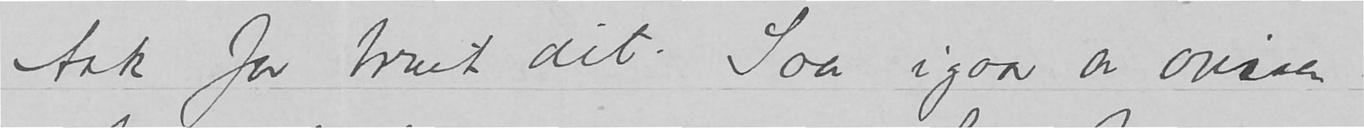tak for brevet dit. Saa igaar av avisen
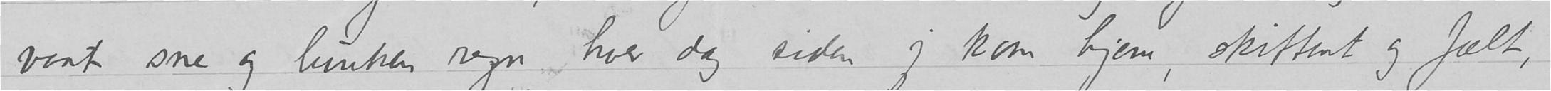vaat sne og lunken regn hver dag siden jeg kom hjem, skittent og fælt,
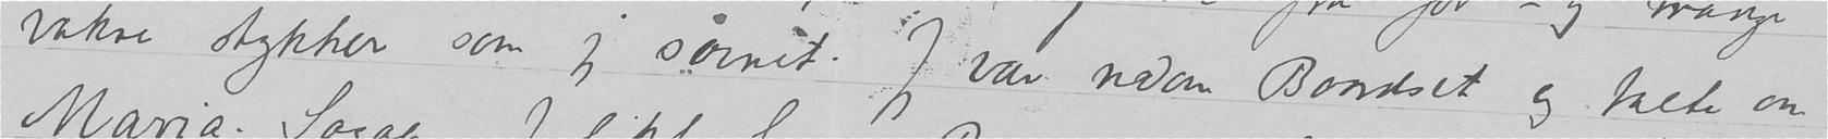vakre stykker som jeg savnet. Jeg var nedom Baardset og talte om
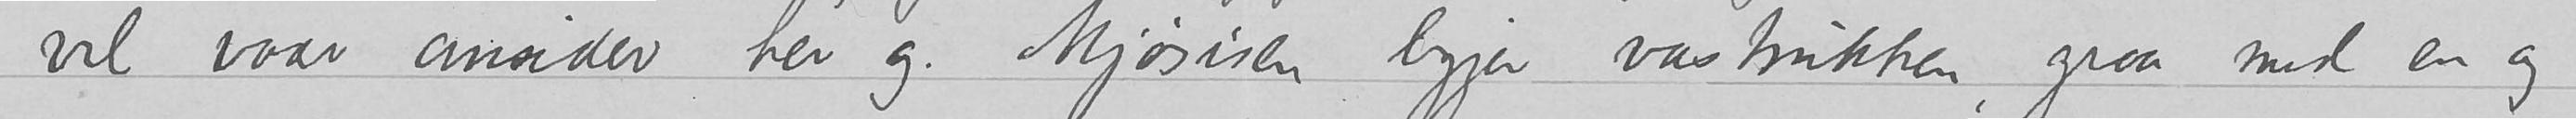vel vaar omsider her og. Mjøsisen ligger vastrukken, graa med en og
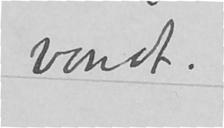vondt.
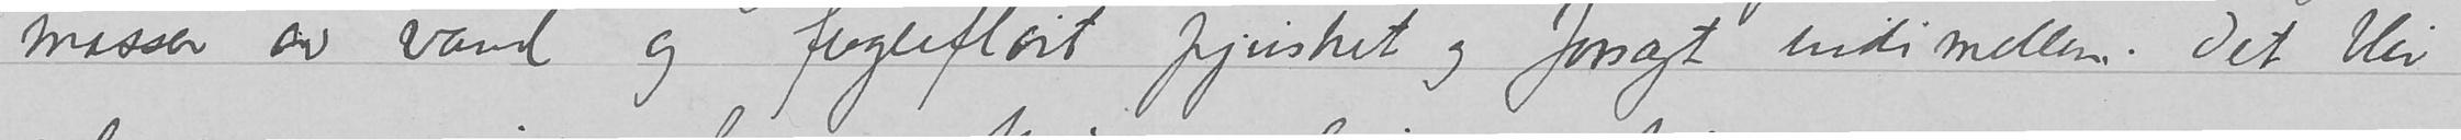masser av vand og fugleflørt pjusket og forsagt indimellem. Det blir
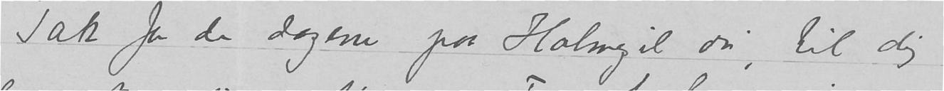Tak for de dagene paa Holmgil du, til dig,
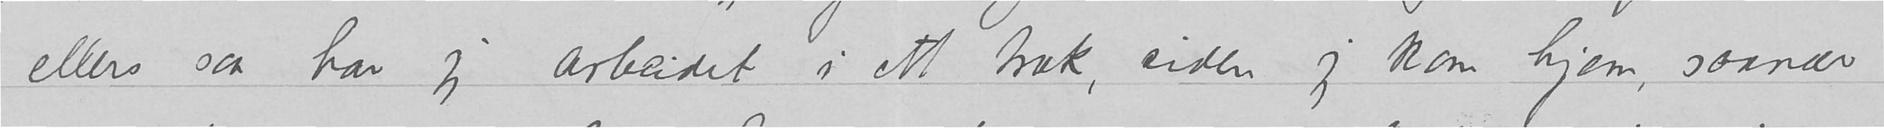ellers saa har jeg arbeidet i ett træk, siden jeg kom hjem, saanær
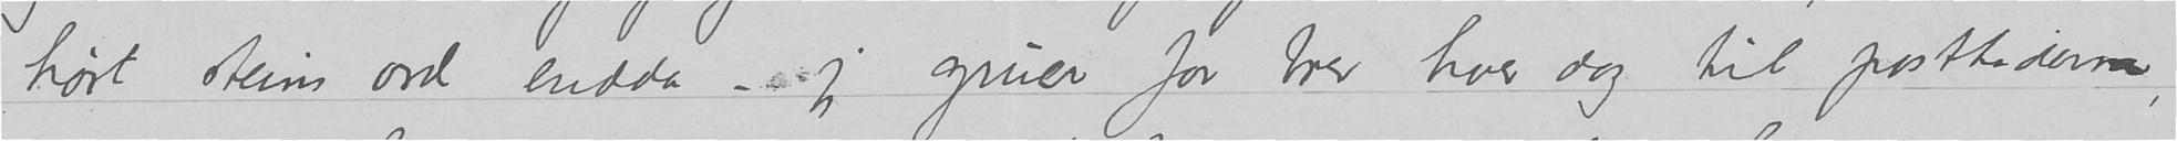hørt steins ord endda – jeg gruer for brev hver dag til posttiderne,
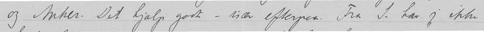og Anker. Det hjalp godt – især efterpaa. Fra S. har jeg ikke
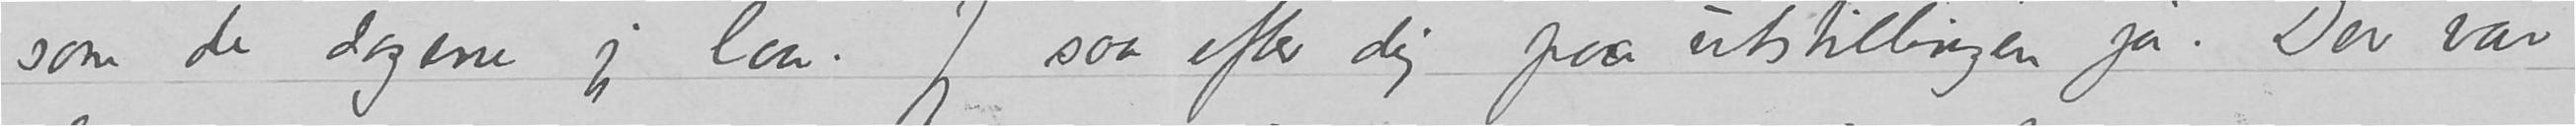som de dagene jeg laa. Jeg saa efter dig paa utstillingen ja. Der var
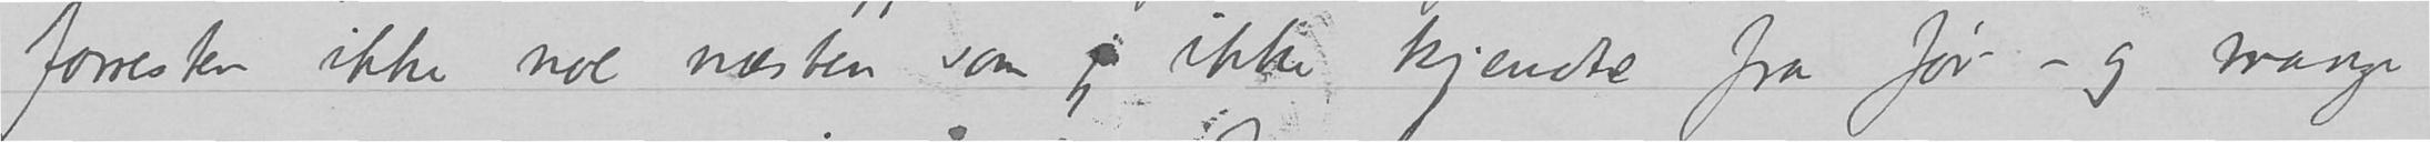forresten ikke noe næsten som jeg ikke kjendte fra før – og mange
